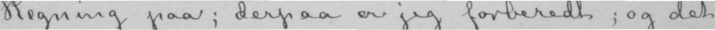Regning paa; derpaa er jeg forberedt; og det
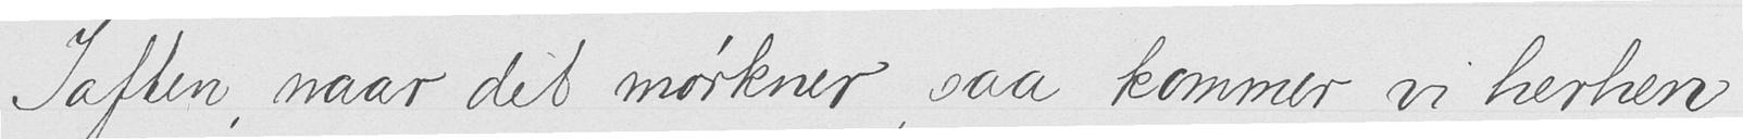Iaften, naar det mørkner, saa kommer vi herhen,
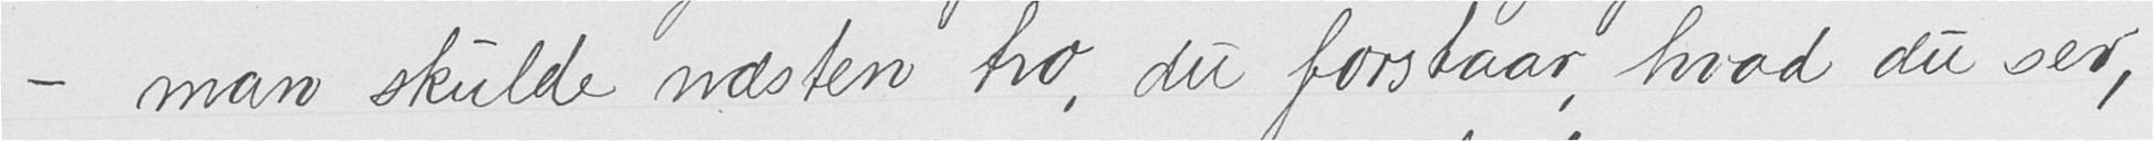- man skulde næsten tro, du forstaar, hvad du ser,
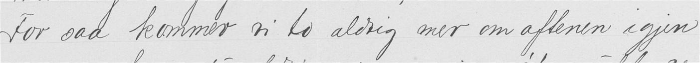For saa kommer vi to aldrig mer om aftenen igjen
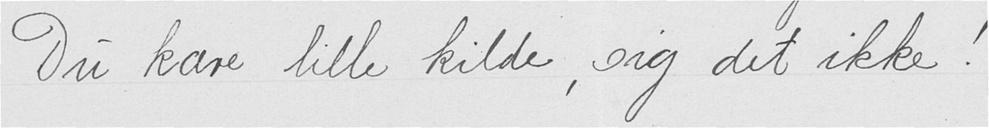- Du kære lille kilde, sig det ikke!
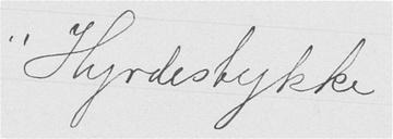"Hyrdestykke"
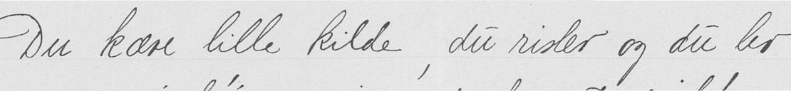Du kære lille kilde, du risler og du ler
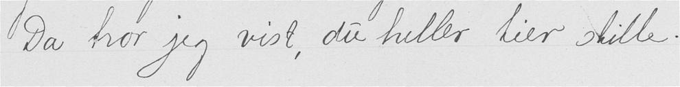Da har jeg vist, du heller tier stille.
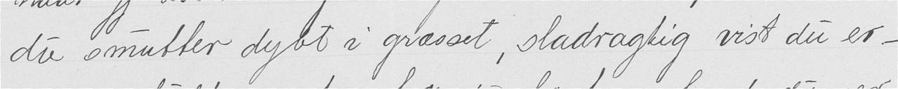du smutter dybt i græsset, sladragtig vist du er -
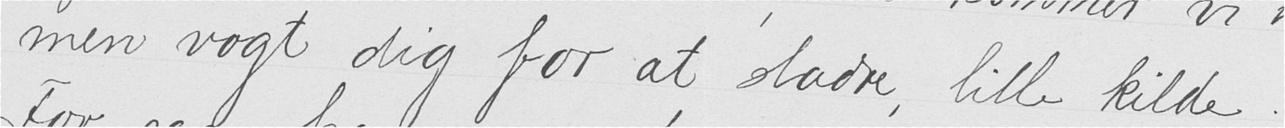men vogt dig for at sladre, lille kilde!
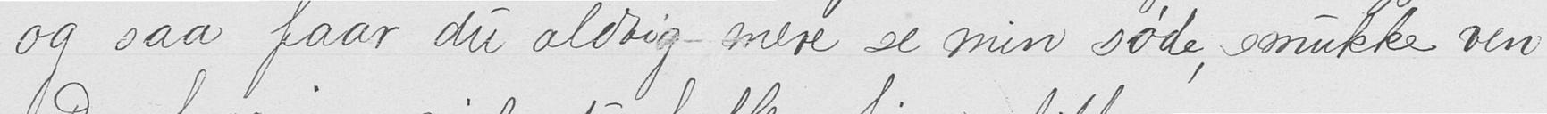og saa faar du aldrig mere se min søde, smukke ven -
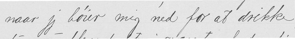naar jeg bøier mig ned for at drikke;
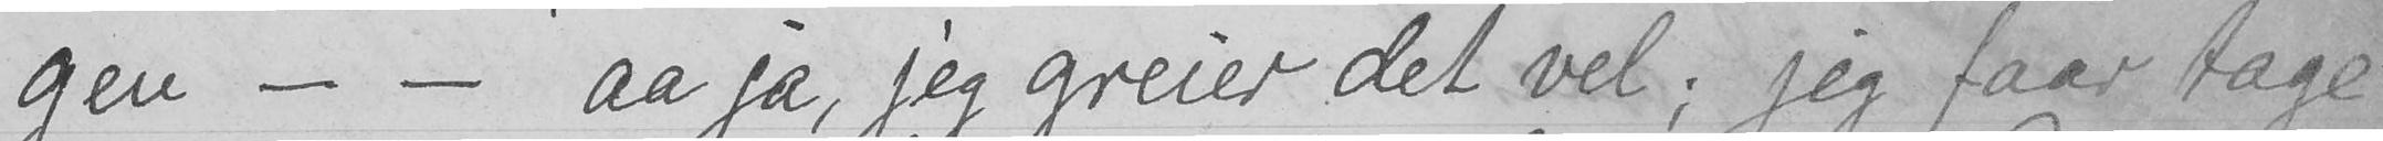gen - - aa ja, jeg greier det vel; jeg faar tage
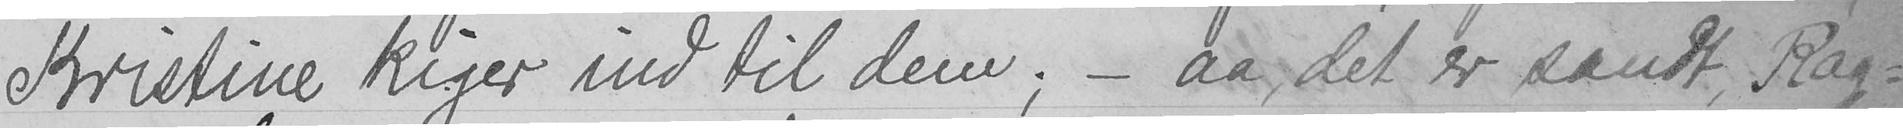Kristine kiger ind til dem; - aa, det er sandt, Rag-
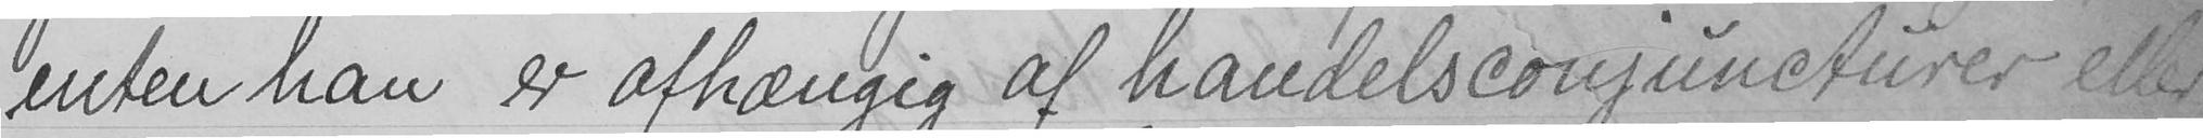enten han er afhængig af handelsconjuncturer eller
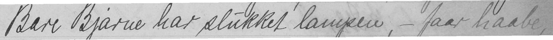Bare Bjarne har slukket lampen, - faar haabe,
